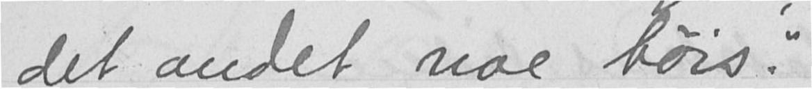det andet noe tøis."
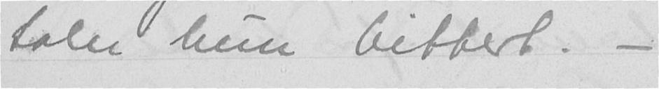taler hun bittert. -
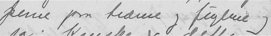perne paa trærne og fuglene og
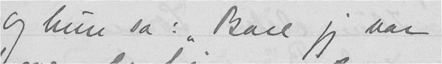Og hun sa: "Bare jeg var
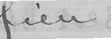fien!
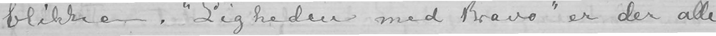blikken. «Ligheden med Bravo» er der alle-
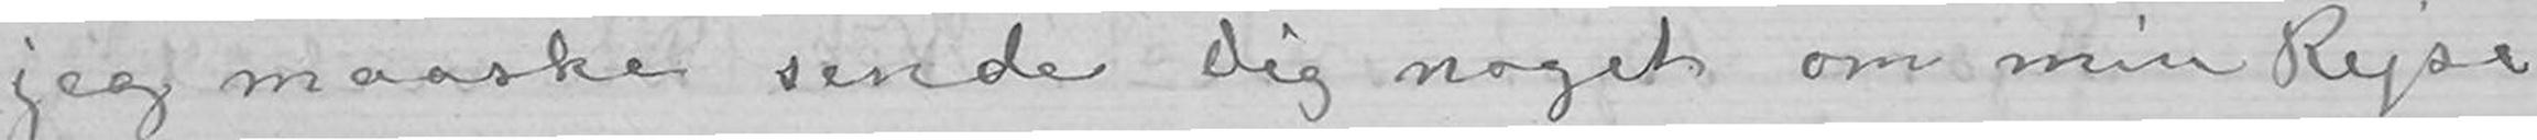jeg maaske sende Dig noget om min Rejse
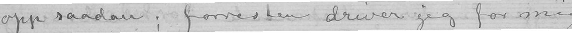opp saadan; forresten driver jeg for mig
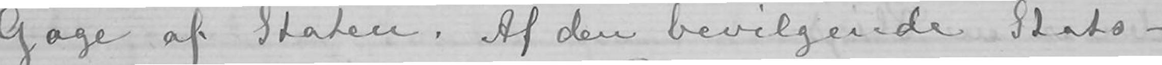Gage af Staten. Af den bevilgende Stats-
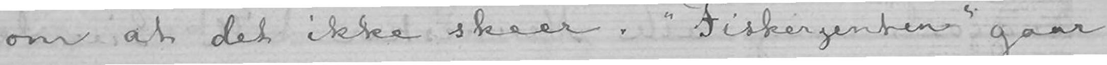om at det ikke skeer. «Fiskerjenten» gaar
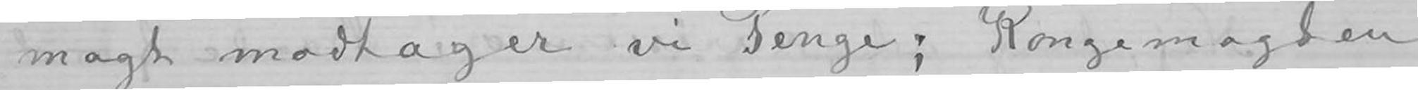magt modtager vi Penge; Kongemagten
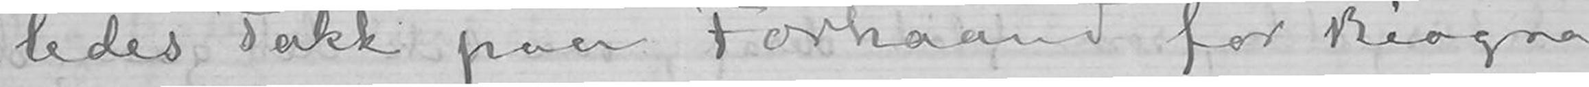ledes Takk paa Forhaand for Biogra-
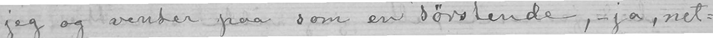jeg og venter paa som en Tørstende,- ja, net-
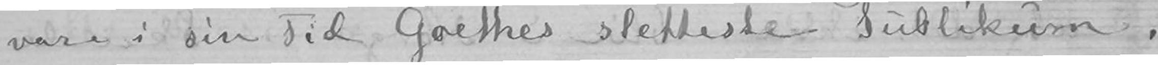vare i sin Tid Goethes sletteste Publikum.
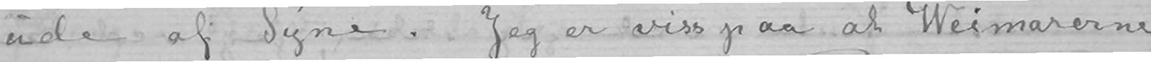ude af Syne. Jeg er viss paa at Weimarerne
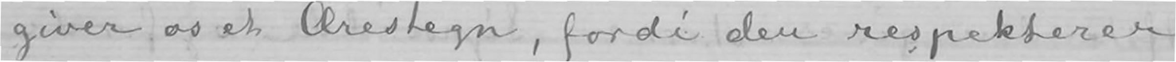giver os et Ærestegn, fordi den respekterer
